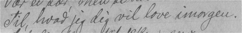Til, hvad jeg dig vil love imorgen.
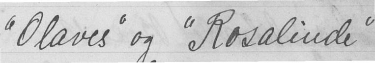"Olaves" og "Rosalinde"
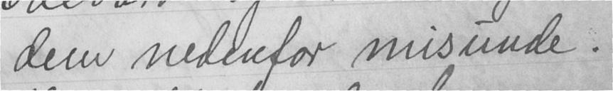dem nedenfor misunde.
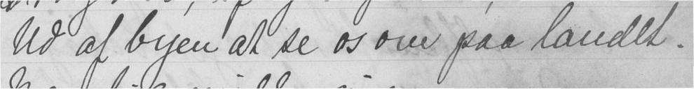Ud af byen at se os om paa landet.
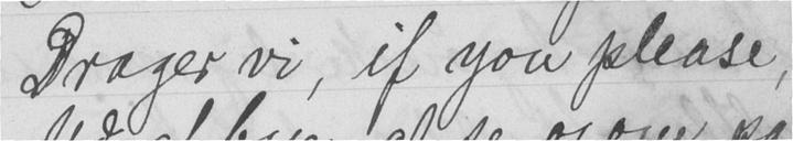Drager vi, if you please,
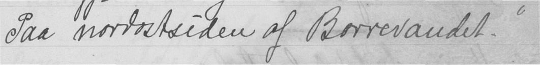Paa nordostsiden af Borrevandet."
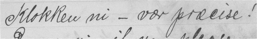Klokken ni - vær præcise!
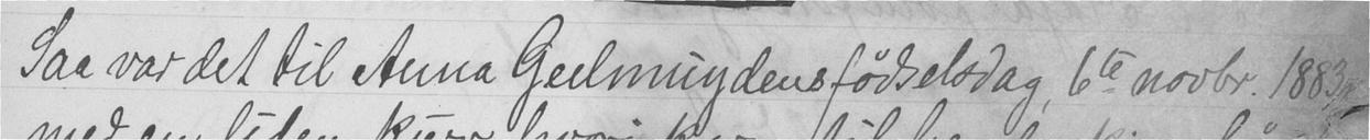Saa var det til Anna Geelmuydens fødselsdag, 6de novbr. 1883
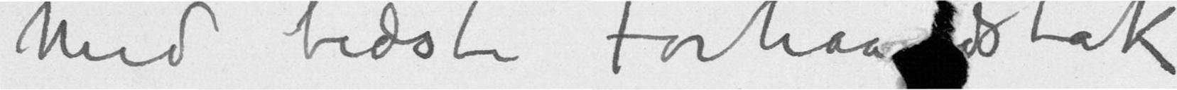Med bedste Forhåndstak
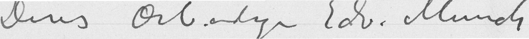Deres ærbødige Edv. Munch
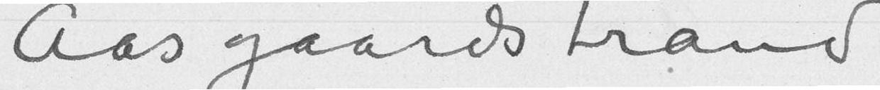Aasgaardstrand
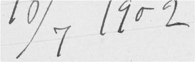18/7 1902
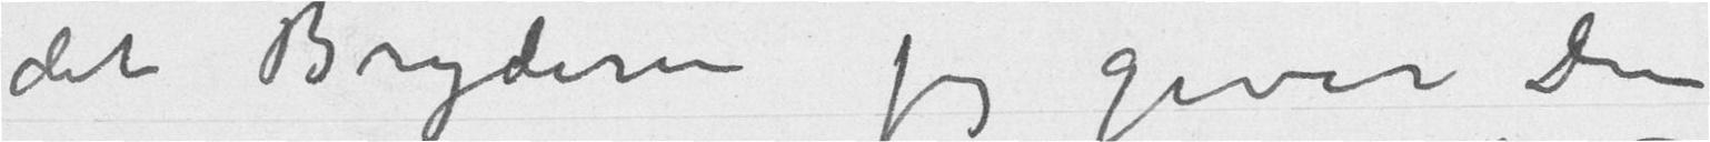det Bryderi jeg giver Dem
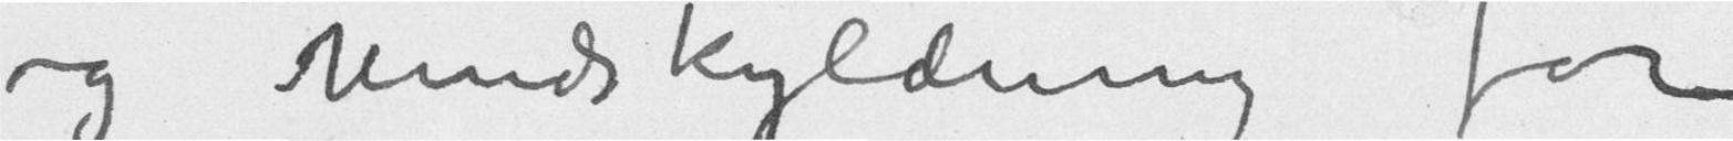og Undskyldning for
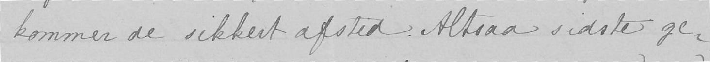kommer de sikkert afsted. Altsaa sidste ge
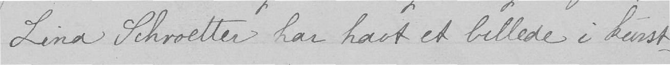Lina Schroetter har havt et billede i kunst-
